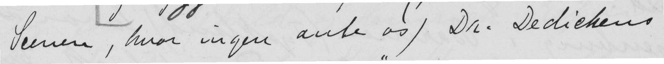Scenen, hvor ingen ante os) Dr. Dedichens
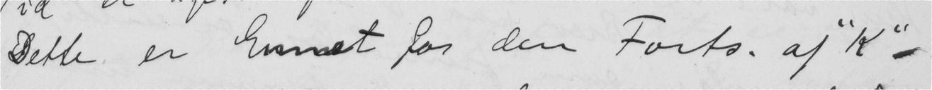Dette er Emnet før den Forts. af "K" -
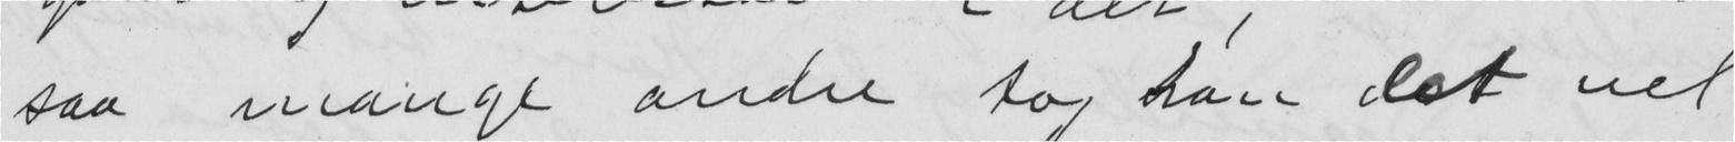saa mange andre tog han det vel
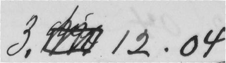3. 12.04
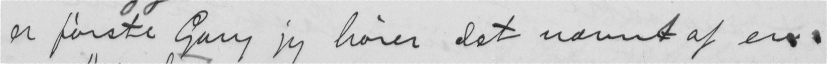er første Gang jeg hører det nævnt af en
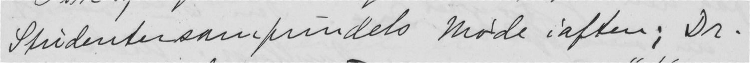Studentersamfundets Møde iaften; Dr.
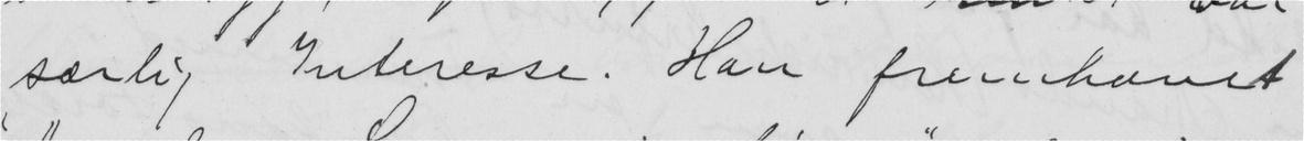særlig Interesse. Han fremhavet
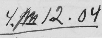4. 12.04
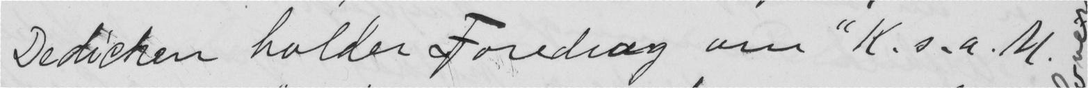Dedichen holder Foredrag om K. s. a. M.
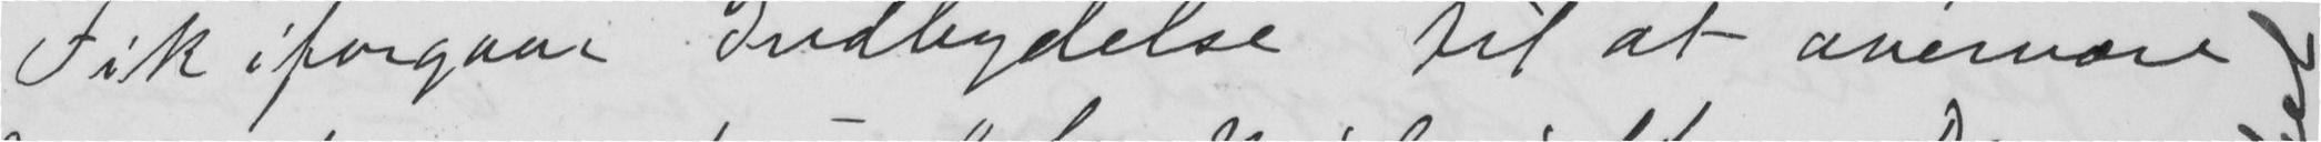Fik iforgaar Indbydelse til at overvære
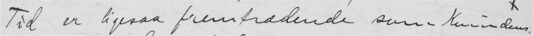Tid er ligesaa fremtrædende som Kvindens.
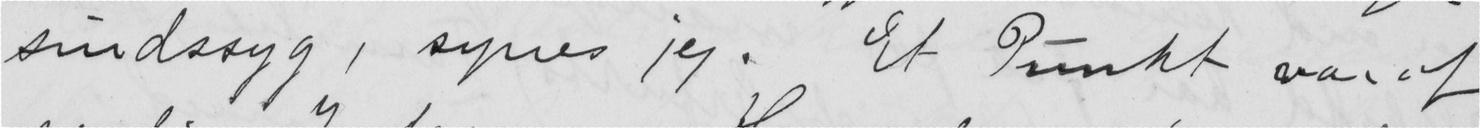sindssyg, synes jeg. Et Punkt var af
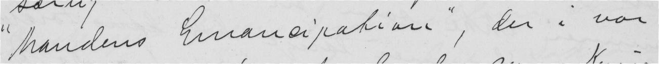"Mandens Emancipation", der i vor
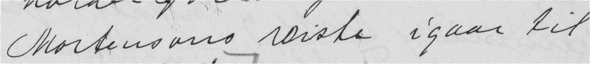Mortensons reiste igaar til
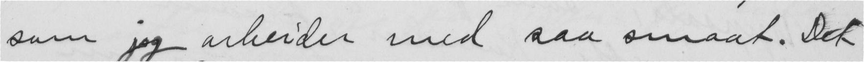som jeg arbeider med saa smaat. Det
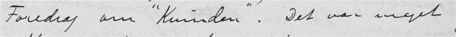Foredrag om "Kvinden". Det var meget
